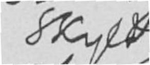Skyld
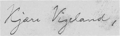Kjære Vigeland,
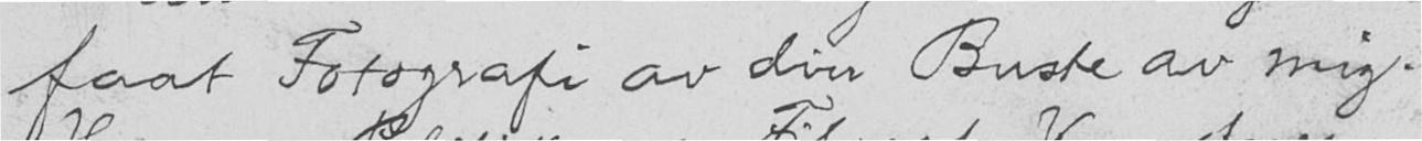faat Fotografi av din Buste av mig.
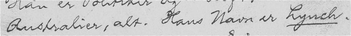Australier, alt. Hans Navn er Lynch.
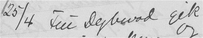25/4 Fru Dybwad gik
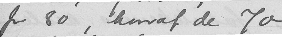for 80, hvoraf de 70
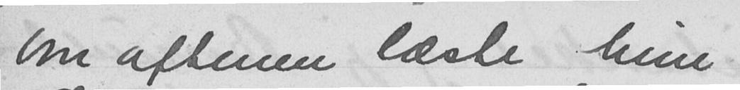Om aftenen læste hun
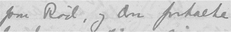paa Rød, og Don fortalte
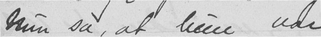hun sa, at hun var
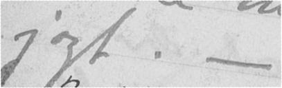jagt. -
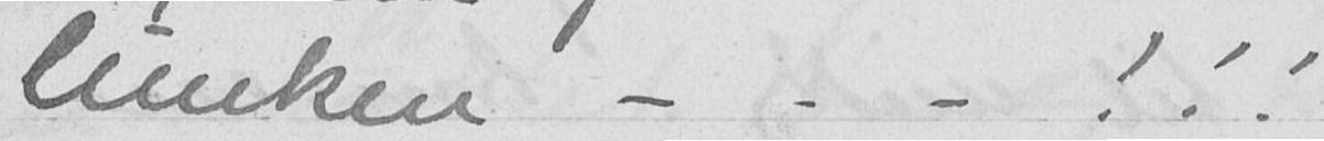lunken - - - !!!
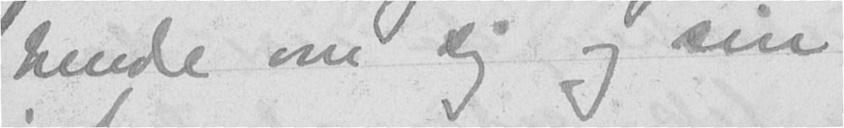hende om sig og sin
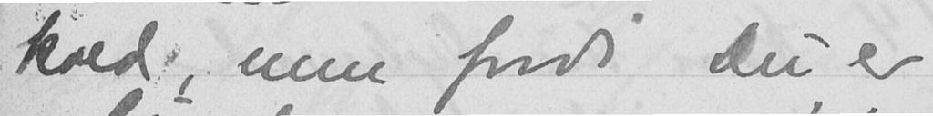kold, men fordi du er
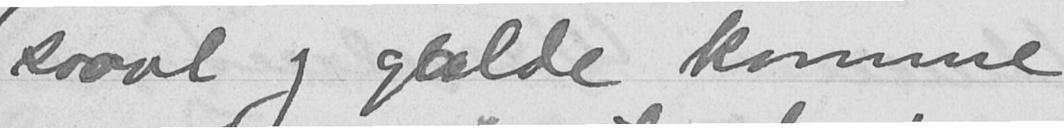svovl og galde komme
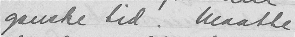ganske tid. Maatte
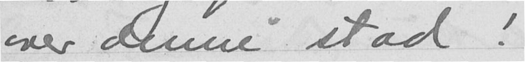over denne stad!
